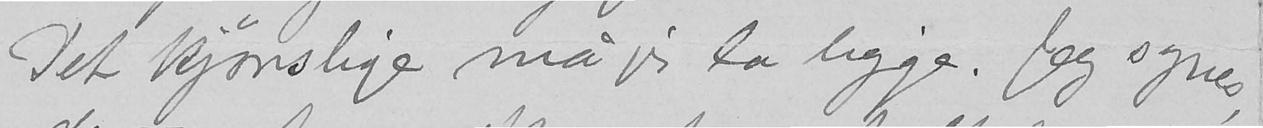Det kjønslige må jeg la ligge. Jeg synes,
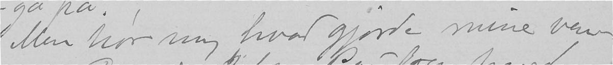Men hør mig hvad gjorde mine ven-
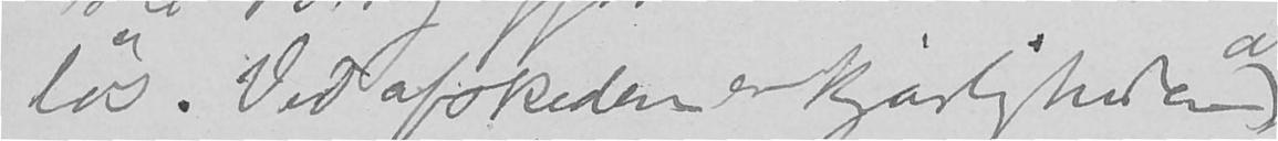løs. Ved afskeden er kjærligheden
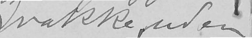vække, uden
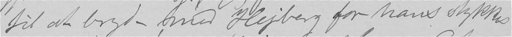til at bryde med Hejberg for hans stykkes
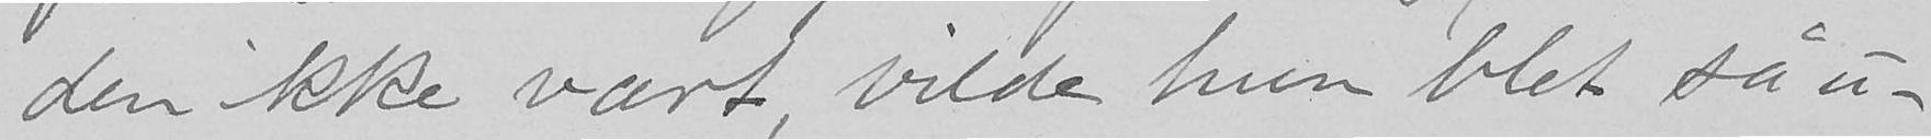den ikke vært, vilde hun blet så u-
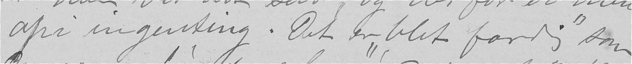opi ingenting. Det er "blit færdig" som
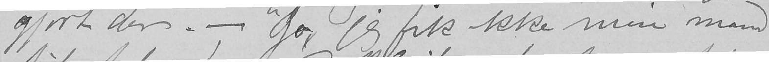gjort der. - Jo, jeg fik ikke min mand
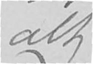alt
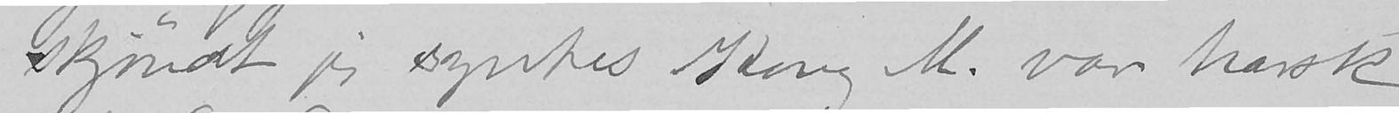skjøndt jeg syntes Kong M. var harsk
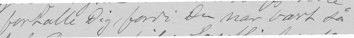fortælle Dig, fordi Du har vært så
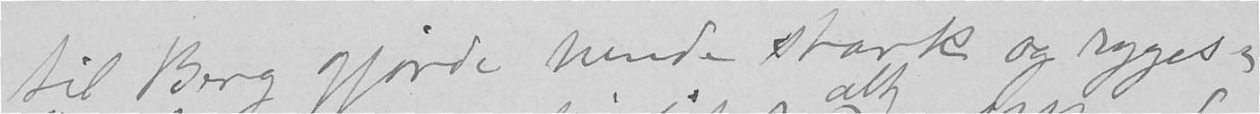til Berg gjorde hende stærk og ryges-
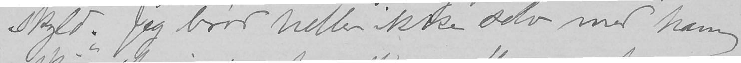skyld. Jeg brød heller ikke selv med ham,
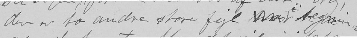der er to andre store fejl med i tegnin-
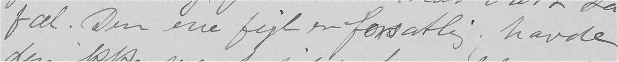fæl. Den ene fejl er forsætlig; havde
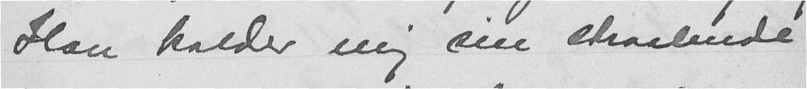Han kalder mig sin straalende
